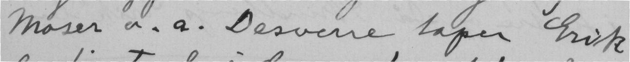Moser a. a. Desverre taper Erik
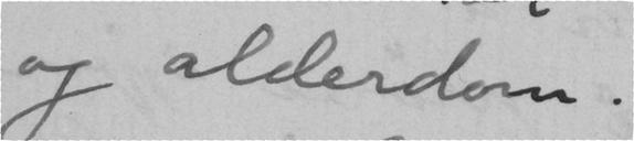og alderdom.
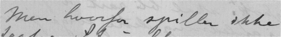Men hvorfor spiller ikke
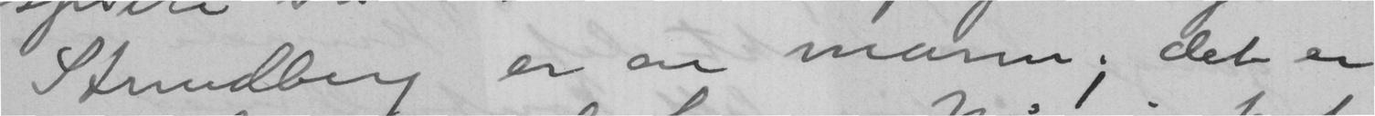Strindberg er en mann; det er
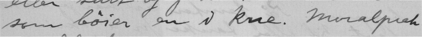som bøier en i kne. Moralprek
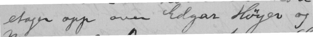etager opp over Edgar Høyer og
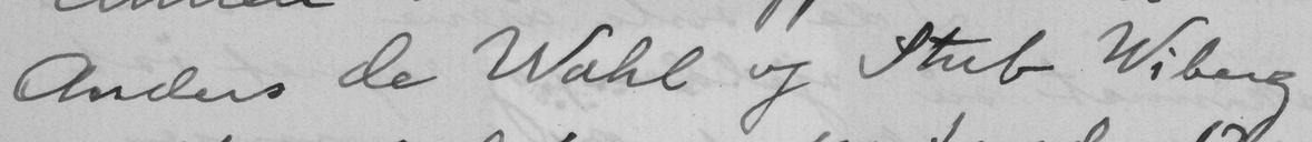Anders de Wahl og Stub Wiberg
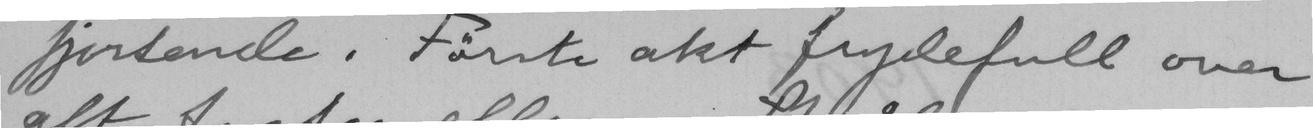fjortende. Første akt frydefull over
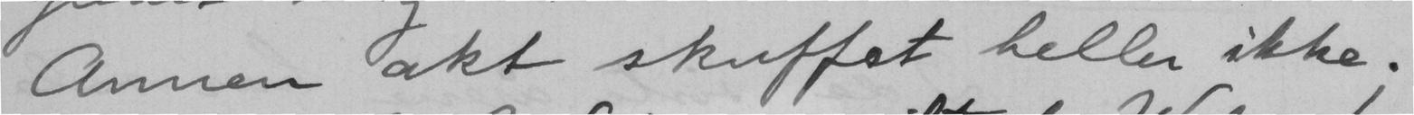Annen akt skuffet heller ikke;
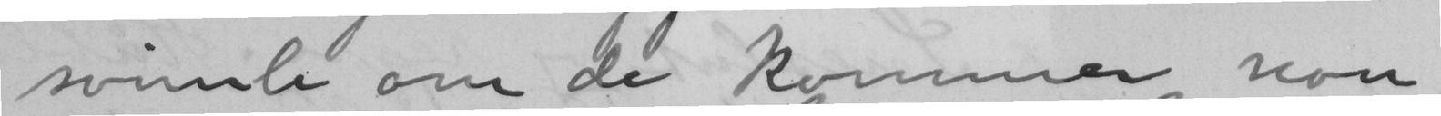svimle om de kommer noen
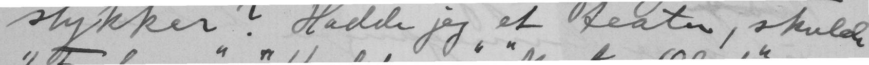stykker? Hadde jeg et teater, skulde
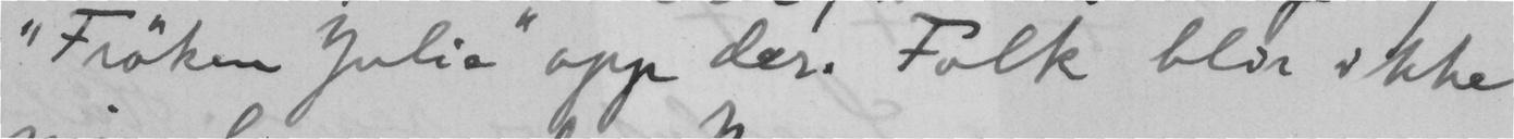"Frøken Julie" opp der. Folk blir ikke
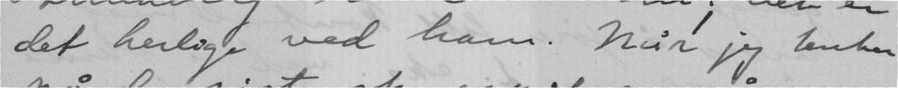det herlige ved ham. Når jeg tenker
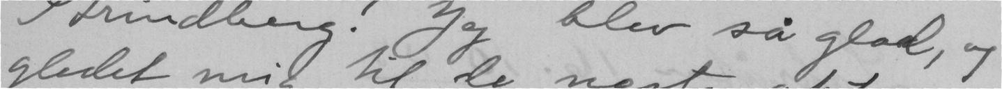Strindberg! Jeg blev så glad, og
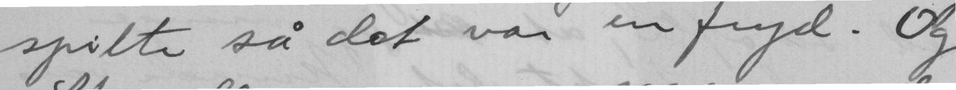spilte så det var en fryd. Og
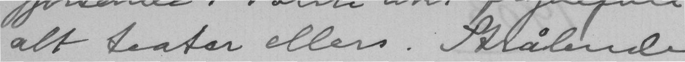alt teater ellers. Strålende
